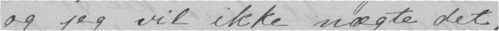og jeg vil ikke nægte det,
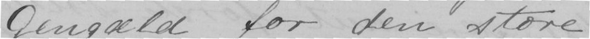Gengæld for den store
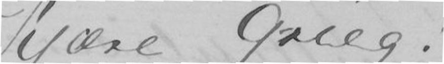Kjære Grieg!
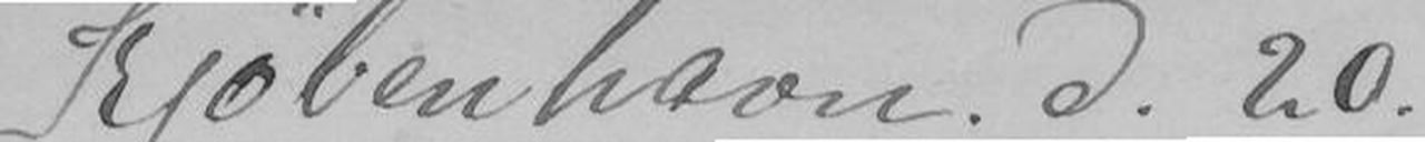Kjøbenhavn. d. 20
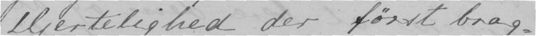Hjertelighed der først brag-
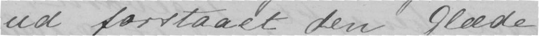ud forstaaet den Glæde
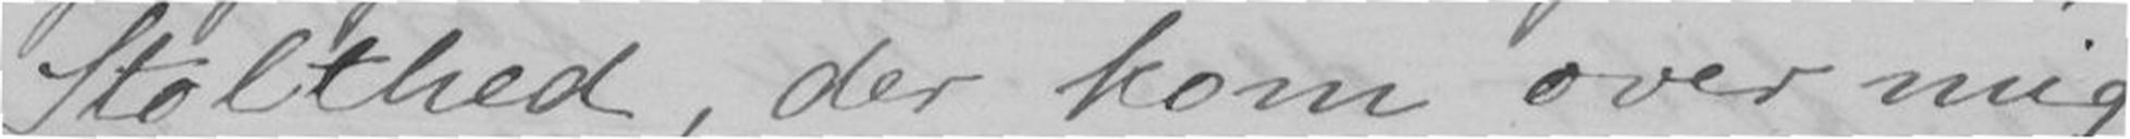Stolthed, der kom over mig,
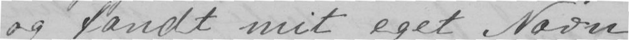og fandt mit eget Navn
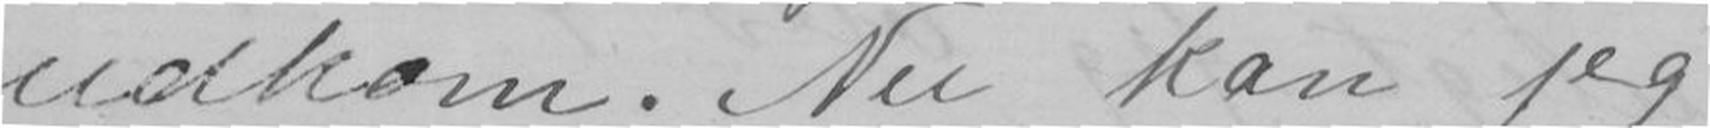udkom. Nu kan jeg
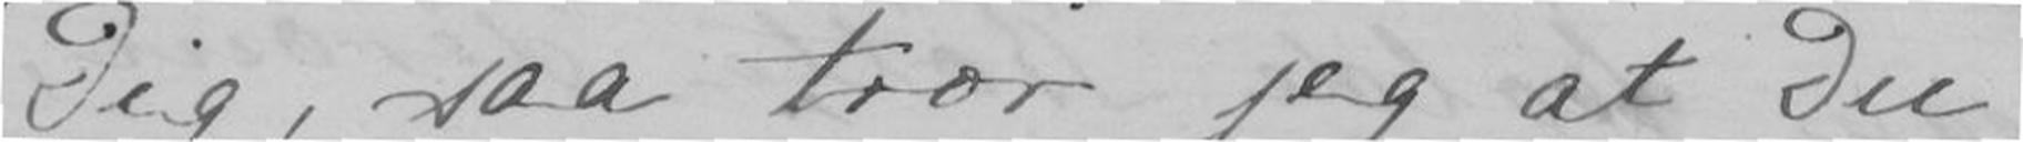Dig, saa tror jeg at Du
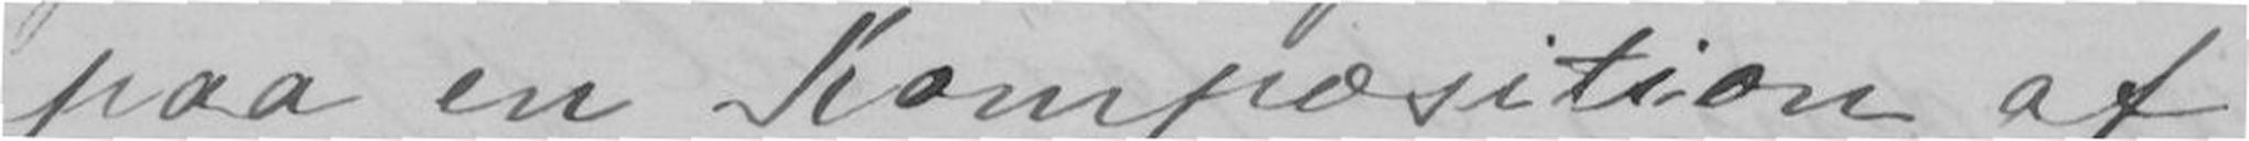paa en Komposition af
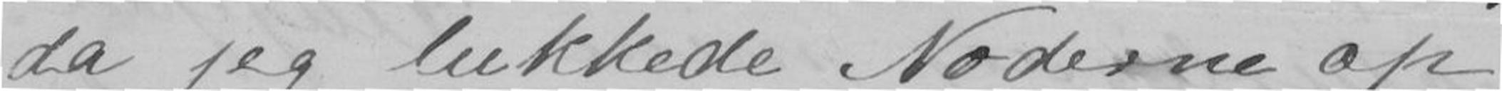da jeg lukkede Noderne op
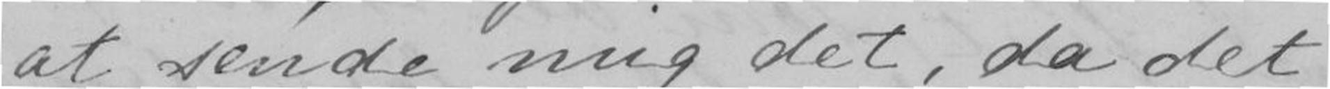at sende mig det, da det
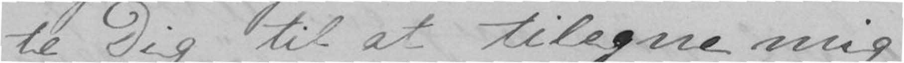te Dig til at tilegne mig
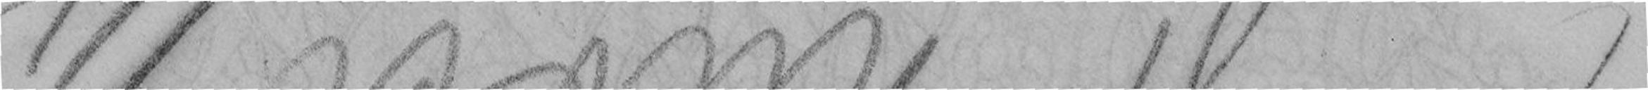Nachs. Kuber 3/12
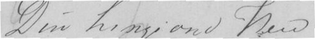Din hengivne Ven
